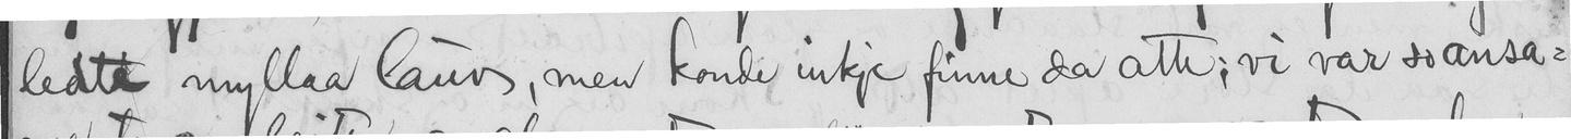ledte myllaa laus, men konde inkje finne da atte; vi var so ansa-
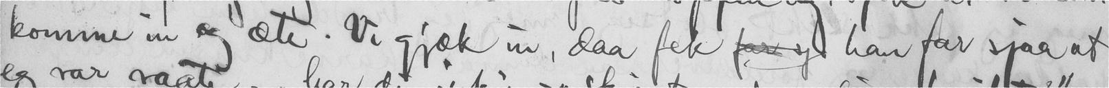komme en og æte. Vi gjæk in, daa fek  han far sjaa at
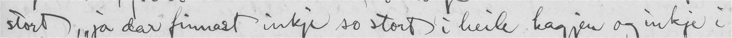stort, "ja dar finnest inkje so stort i heile hagjen og inkje i
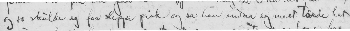og so skulde eg faa sleppe pisk og sa han endaa eg mest tårde hat
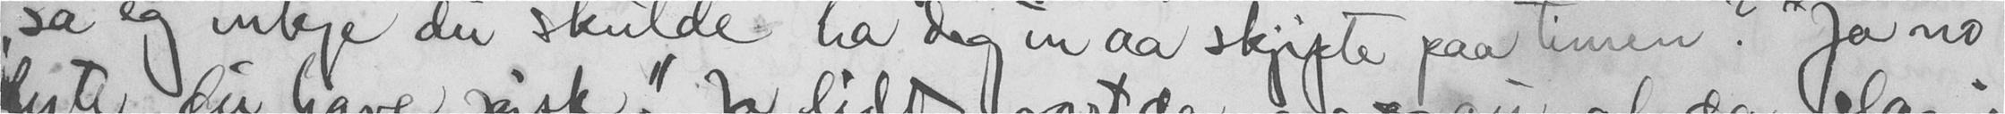sa eg inkje du skulde ha deg en aa skjifte paa timen?" Ja no
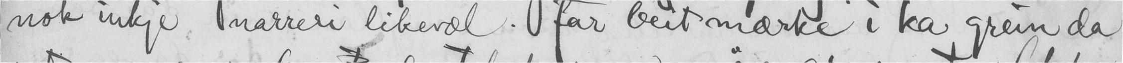nok inkje, narreri likevæl. Far beit mærke i ka grein da
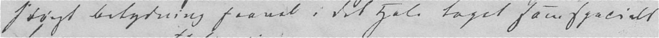størst Betydning saavel i det Hele taget som specielt
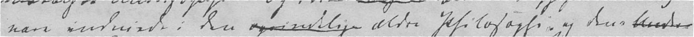vare indviede i den ældre Philosophie og dens
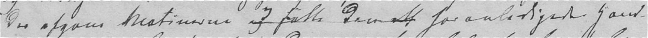der afgav Nationerne og der foranledigede Hand-
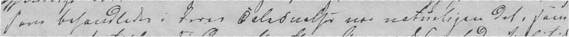som behandledes i deres Taleøvelser var naturligen det, som
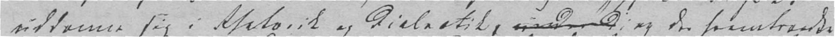uddanne sig i Rethorik og Dialectik, og der fremtraadte
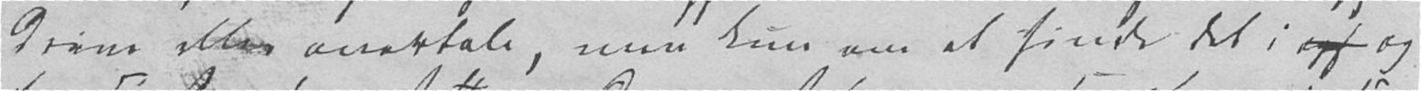drive eller overtale, men kun om at finde det i og
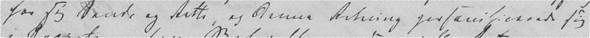for sig Sande og Rette og denne Retning personificerede sig
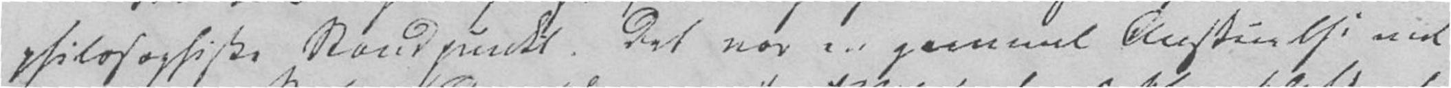philosophiske Standpunkt. Det var en gammel Anskuelse end
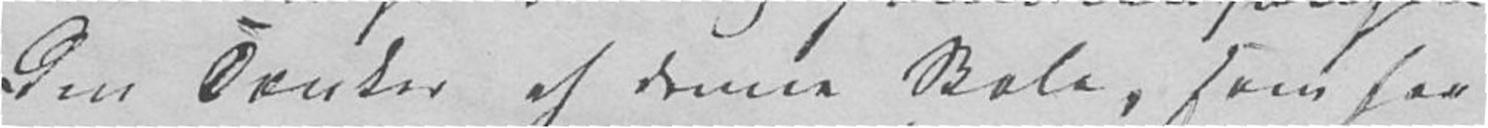Den Tænker af denne Skole, som har
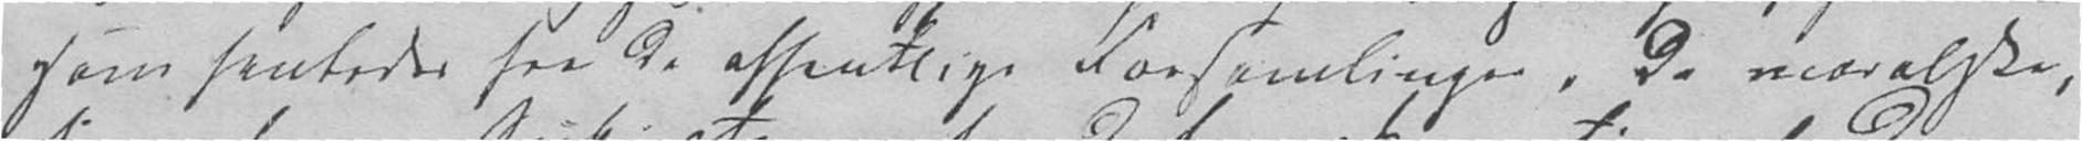som hentedes fra de offentlige Forsamlinger, de moralske,
